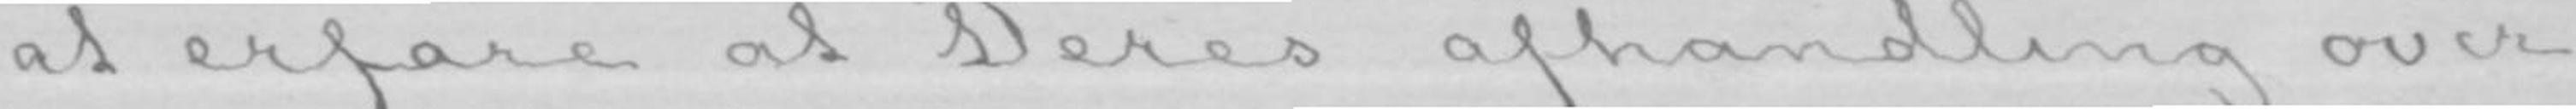at erfare at Deres afhandling over
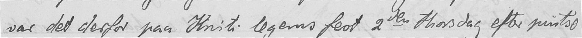var det derfor paa Kristi legems fest 2den thorsdag efter pintse
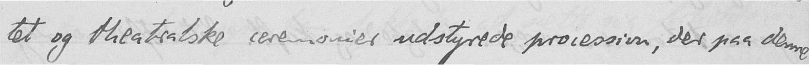tet og theatralske ceremonier udstyrede procession, der paa denne
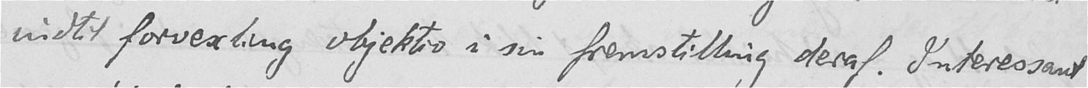indtil forvexling objektiv i sin fremstilling deraf. Interessant
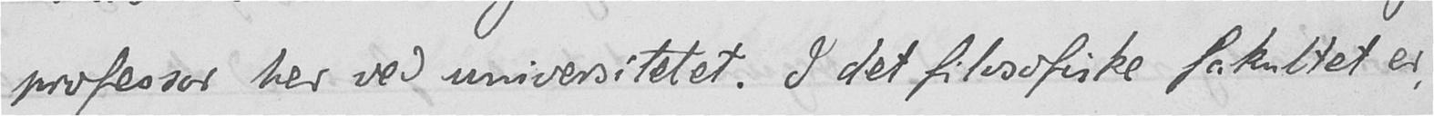professor her ved universitetet. I det filosofiske fakultet er,
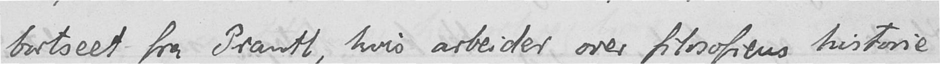bortseet for Prandtl, hvis arbeider over filosofiens historie
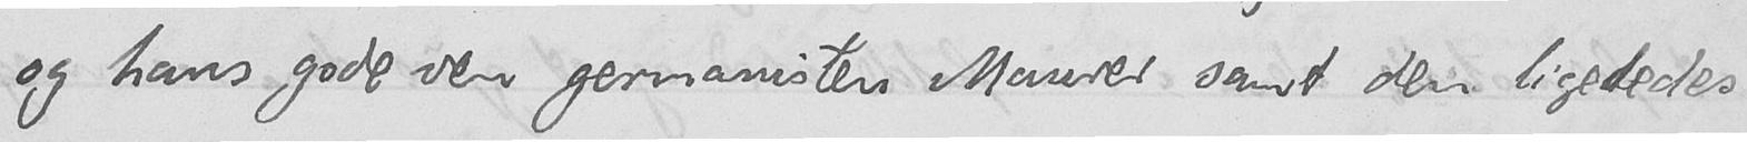og hans gode ven germanisten Maurer, samt den ligeledes
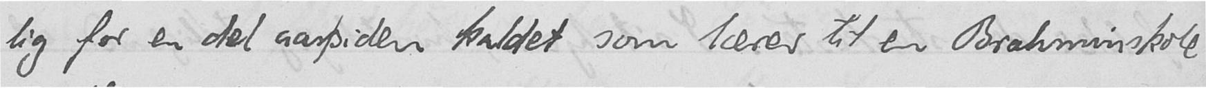lig for en del aar siden kaldet som lærer til en Brahminskole
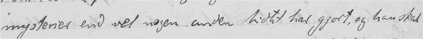mysterier end vel nogen anden hidtil har gjort, og han skal
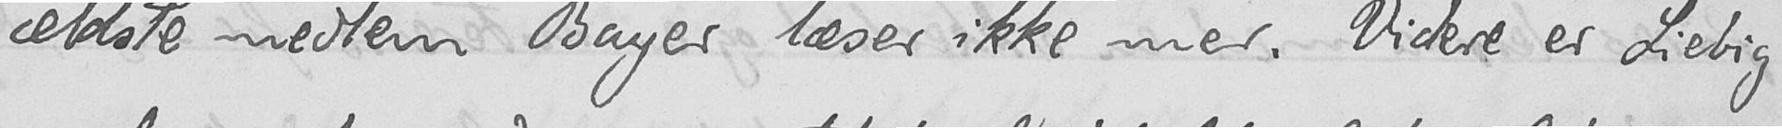ældste medlem Bayer læser ikke mer. Videre er Liebig
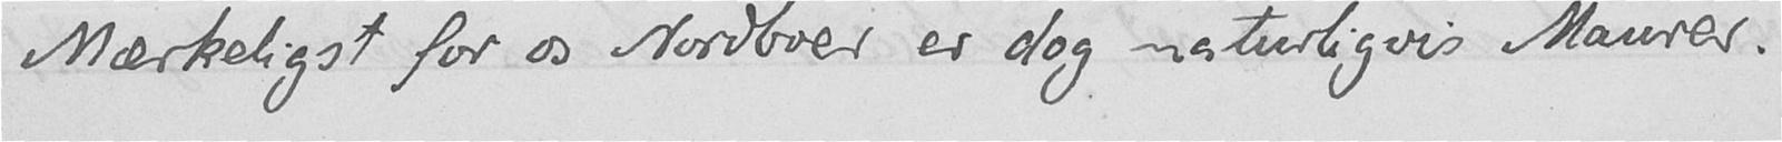Mærkeligst for os Nordboer er dog naturligvis Maurer.
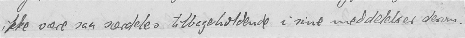ikke være saa særdeles tilbageholdende i sine meddelelser derom.
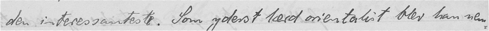den interessanteste. Som yderst lærd orientalist blev han nem-
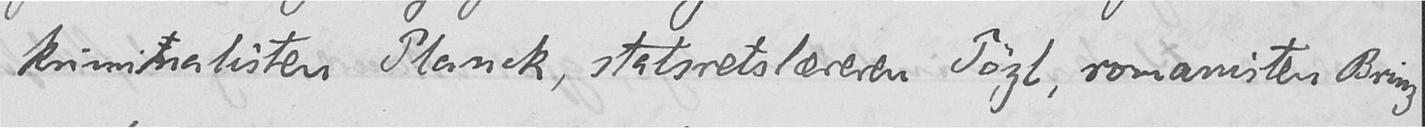kriminalisten Planck, statsretslæreren Pözl, romanisten Brinz
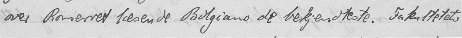over Romerret læsende Bolgiano de bekjendteste. Fakultetets
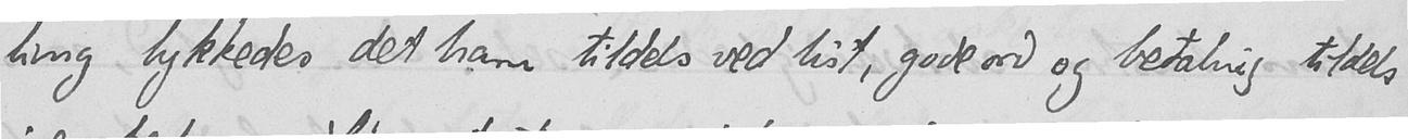ling lykkedes det ham tildels ved list, gode ord og betaling tildels
